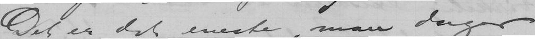Det er det eneste, man duger
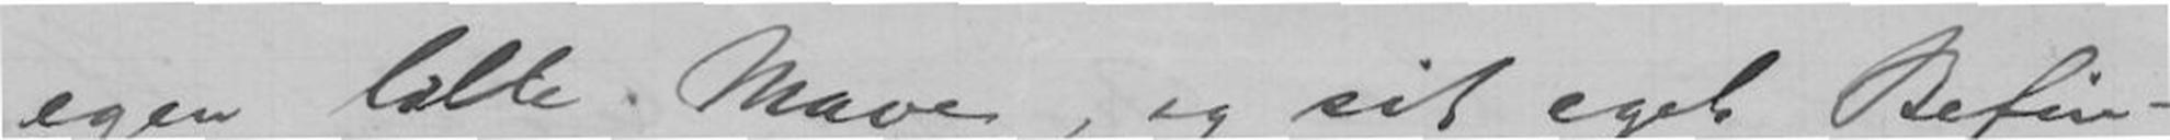egen lille Mave, og sit eget Befin-
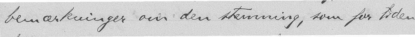bemærkninger om den stemning, som for tiden
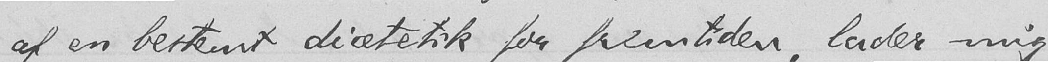af en bestemt diætetik for fremtiden, lader mig
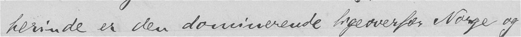herinde er den dominerende ligeoverfor Norge og
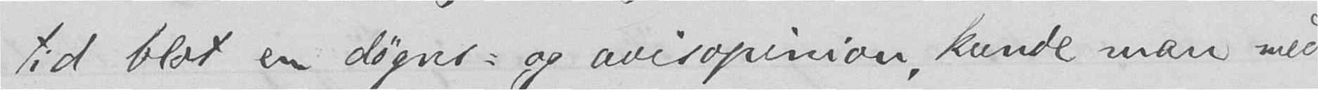tid blot en døgns- og avisopinion, kunde man med
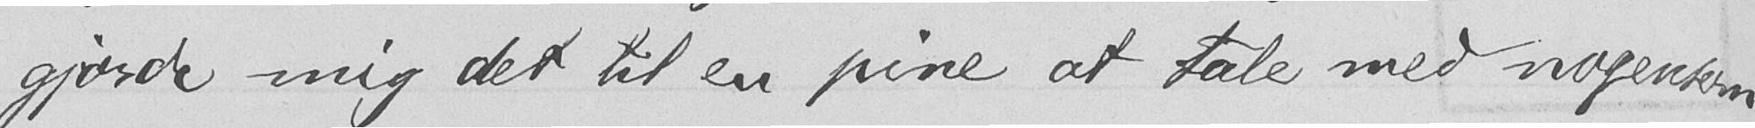gjorde mig det til en pine at tale med nogensom-
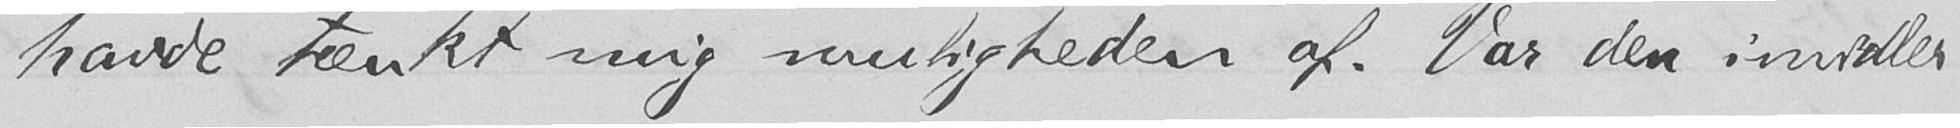havde tænkt mig muligheden af. Var den imidler-
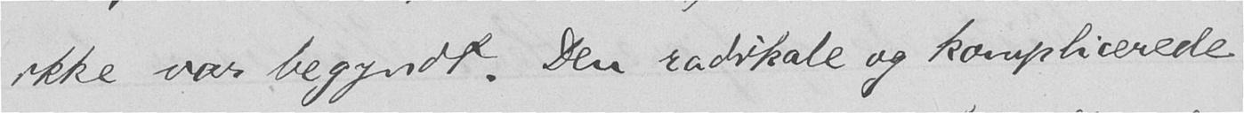ikke var begyndt. Den radikale og komplicerede
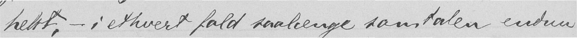helst, - i ethvert fald saalænge samtalen endnu
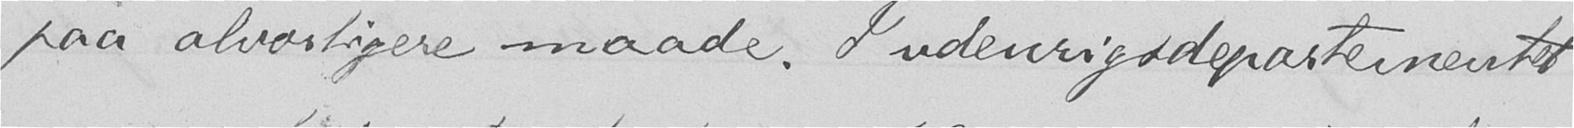paa alvorligere maade. I udenrigsdepartementet
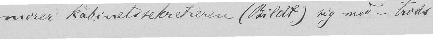morer kabinetssekretæren (Bildt) sig med - trods
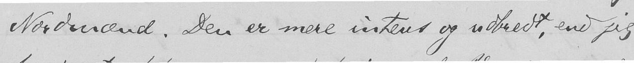Nordmænd. Den er mere intens og udbredt, end jeg
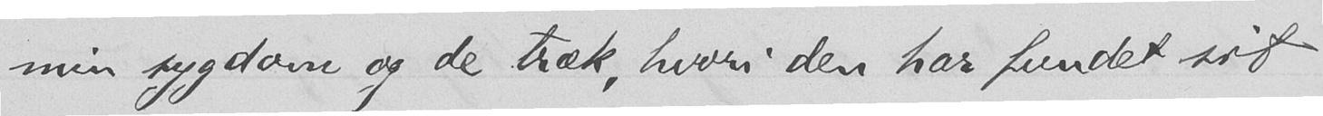min sygdom og de træk, hvori den har fundet sit
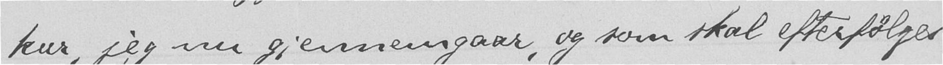kur, jeg nu gjennemgaar, og som skal efterfølges
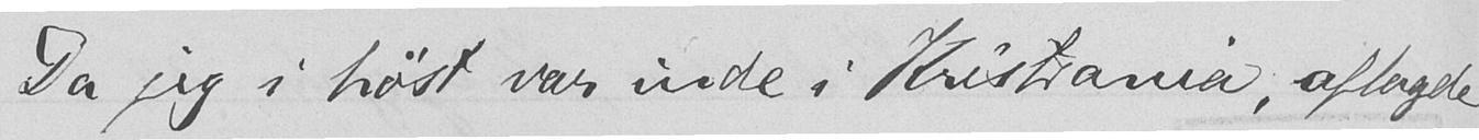Da jeg i høst var inde i Kristiania, aflagde
